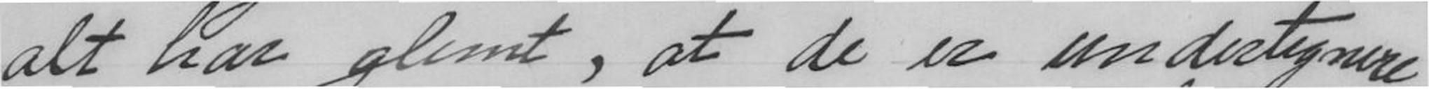alt har glemt, at de er undertegnere
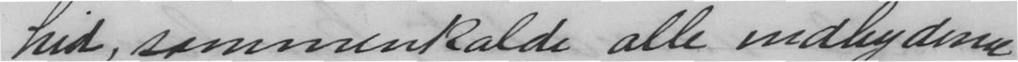hid, sammenkalde alle indbyderne
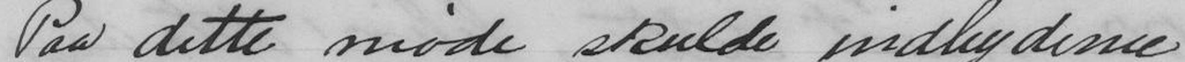Paa dette møde skulde indbyderne
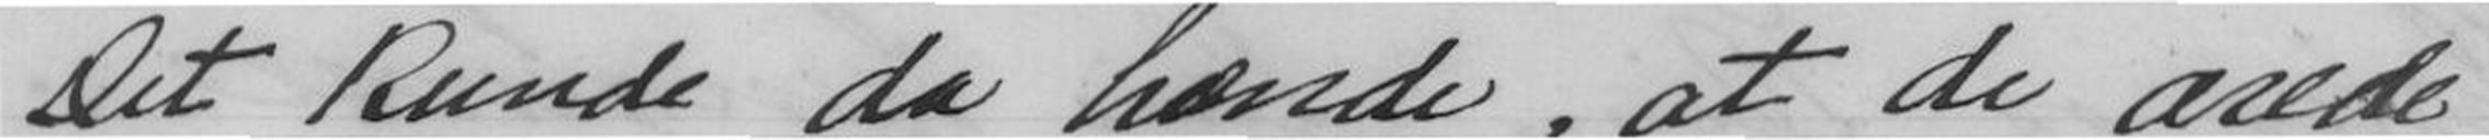Det kunde da hænde, at de ærede
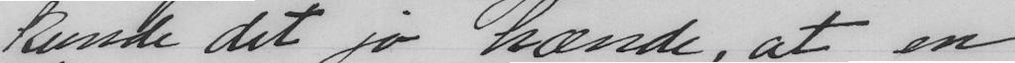kunde det jo hænde, at en
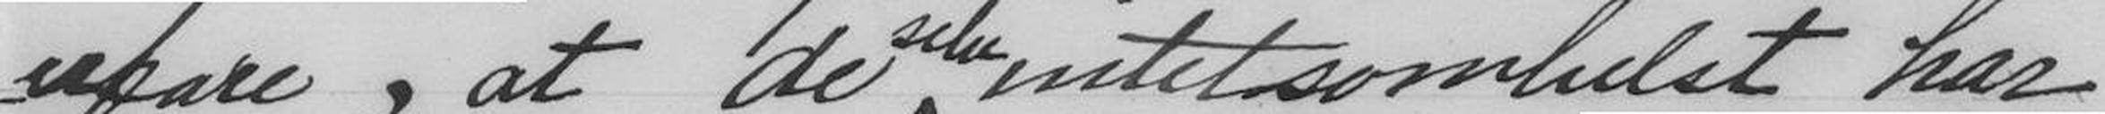erfare, at de selv intetsomhelst har
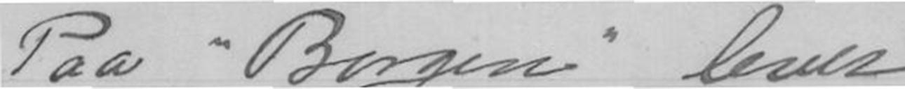Paa "Borgen" lever
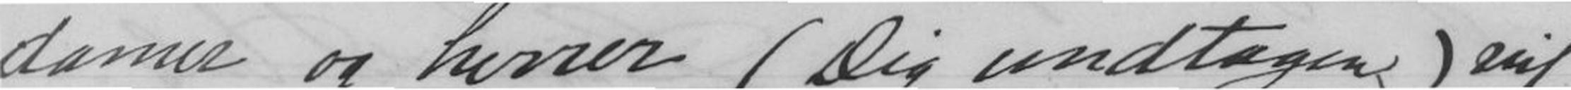damer og herrer (Dig undtagen) vil
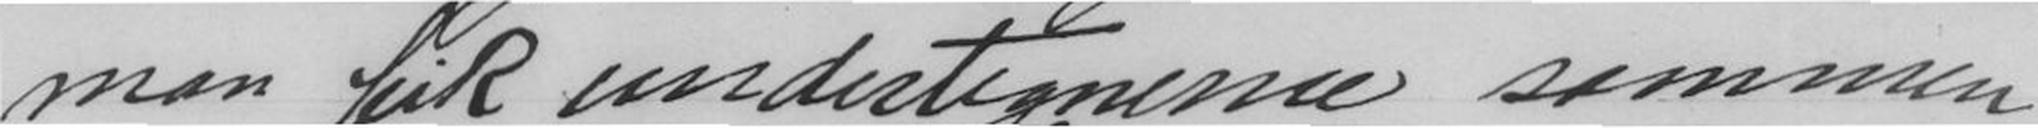man fik undertegnerne sammen,
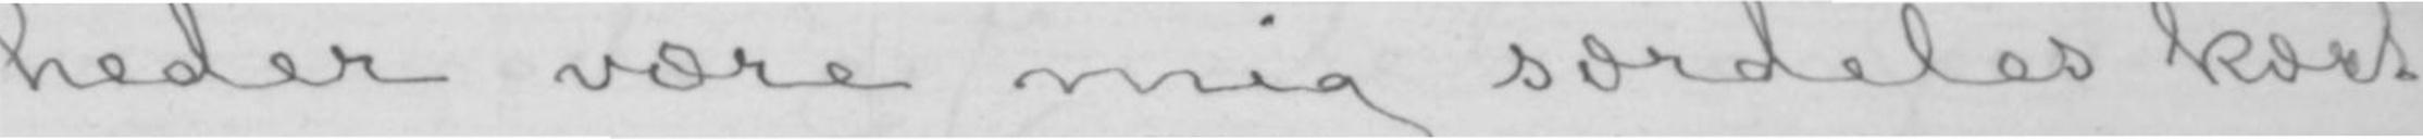heder være mig særdeles kært
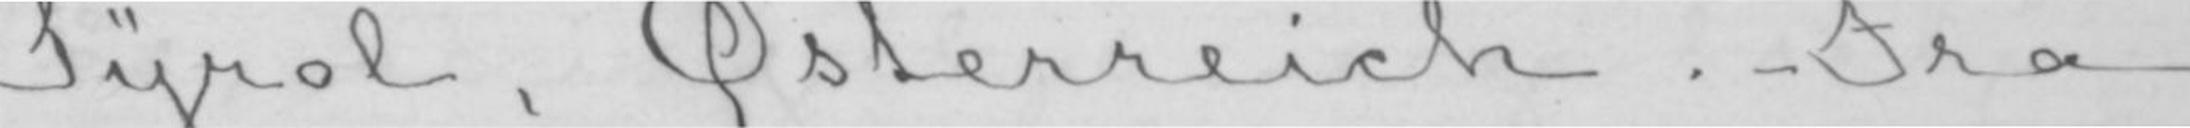Tyrol, Österreich.- Fra
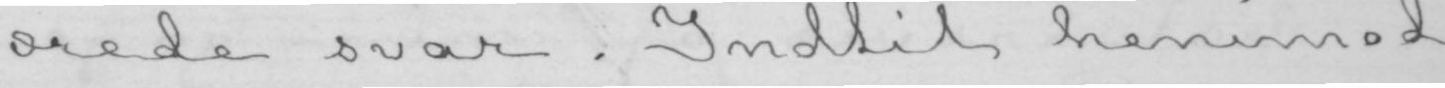ærede svar. Indtil henimod
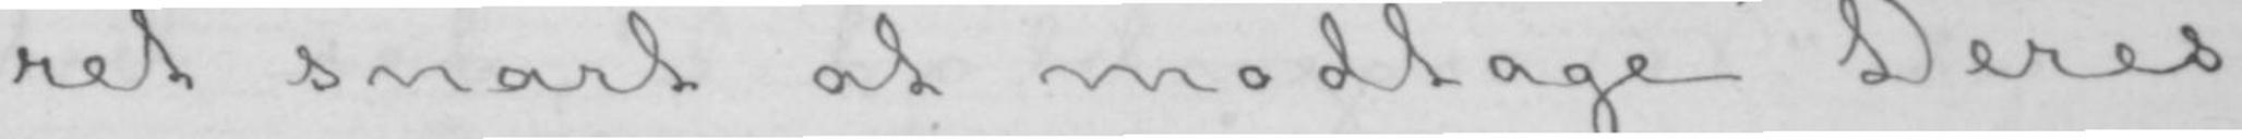ret snart at modtage Deres
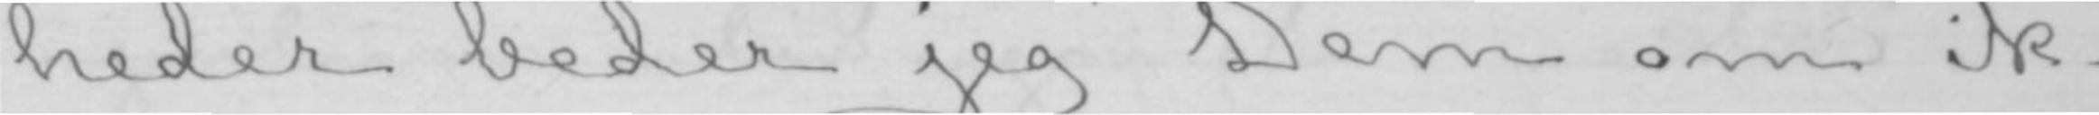heder beder jeg Dem om ik-
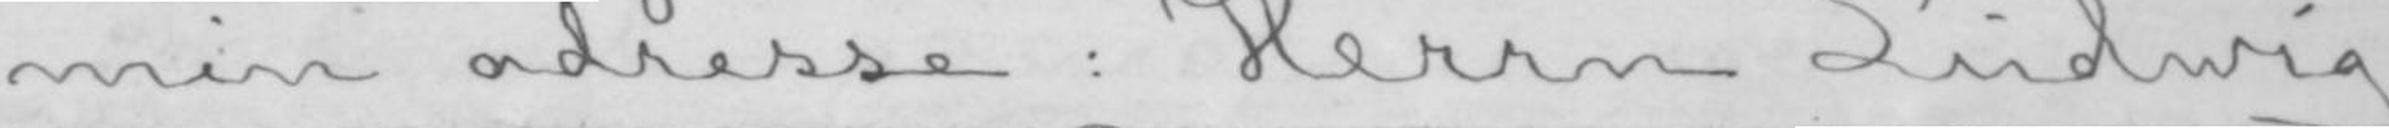min adresse: Herrn Ludwig
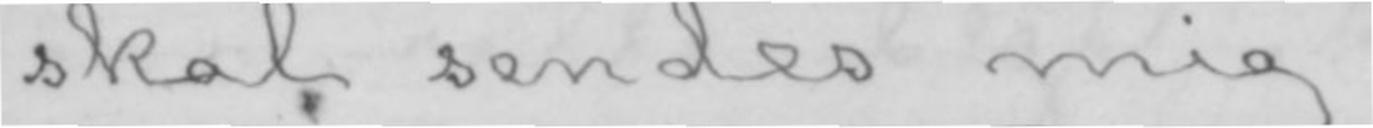skal sendes mig.
